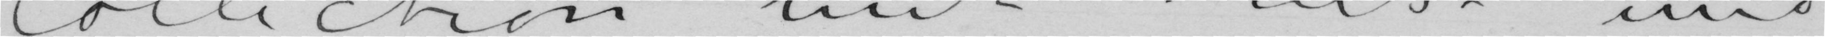Collection mit Preis- und
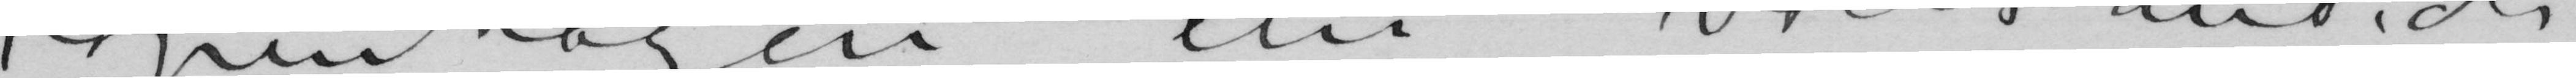Kopenhagen ein vollstandich
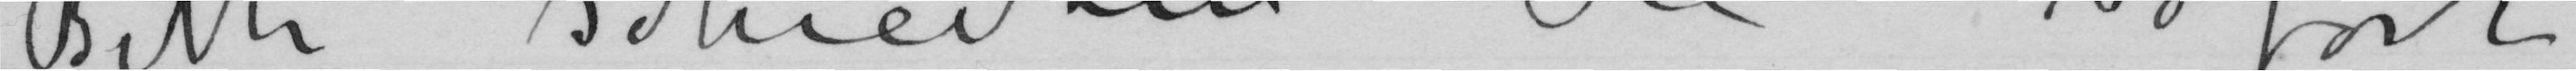Bitte schiecken Sie sofort
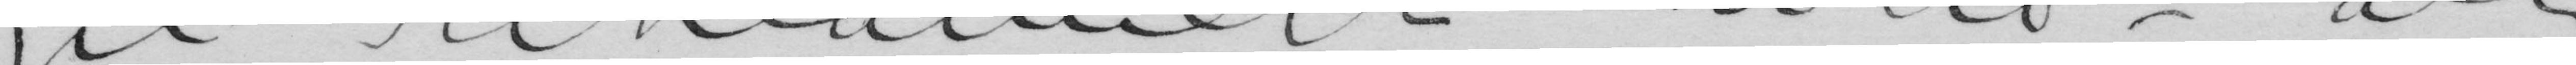gu reklamiert wird – alz
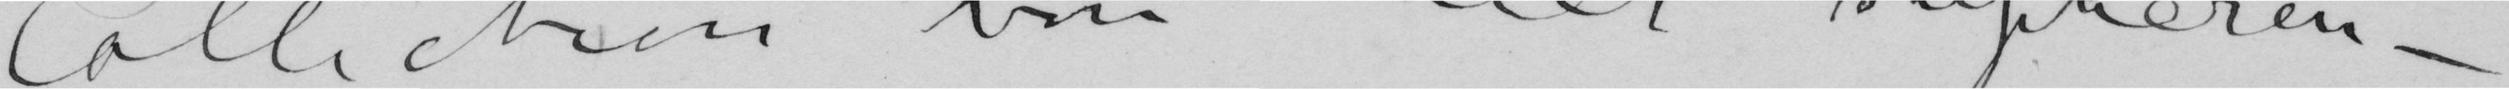Collection von hier suplieren –
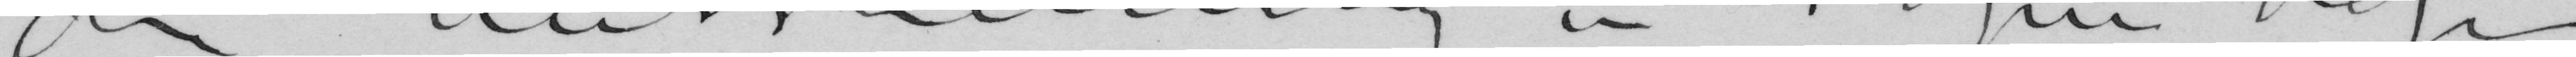die Ausstellung in Kopenhagen
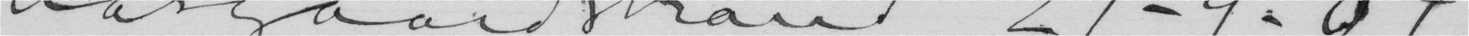Aasgaardstrand 29-9-04
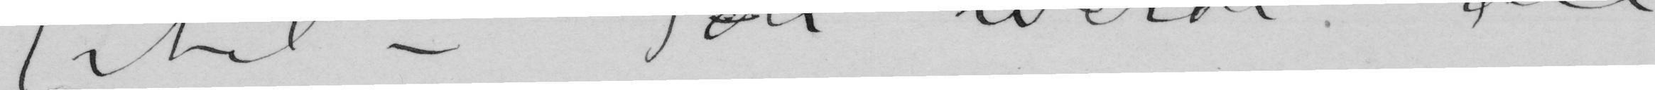Titel – Ich werde der
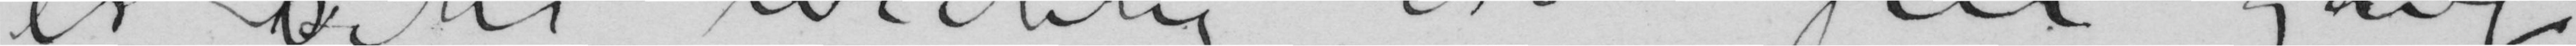es sehr wichig ist fur ganz
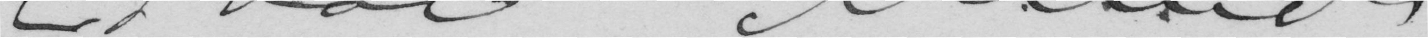Edvard Munch
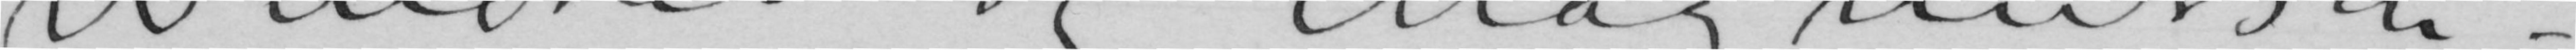Winkel og Magnussen –
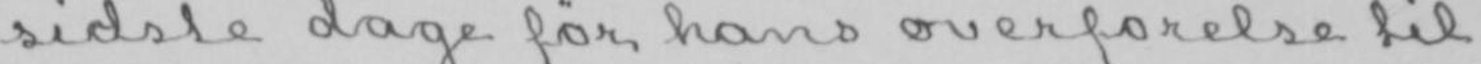sidste dage før hans overførelse til
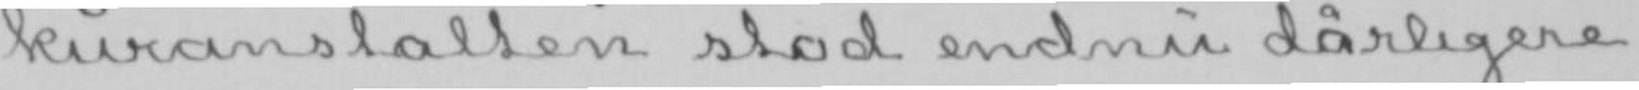kuranstalten stod endnu dårligere
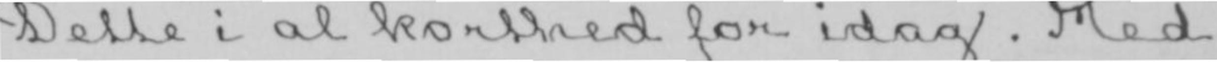Dette i al korthed for idag. Med
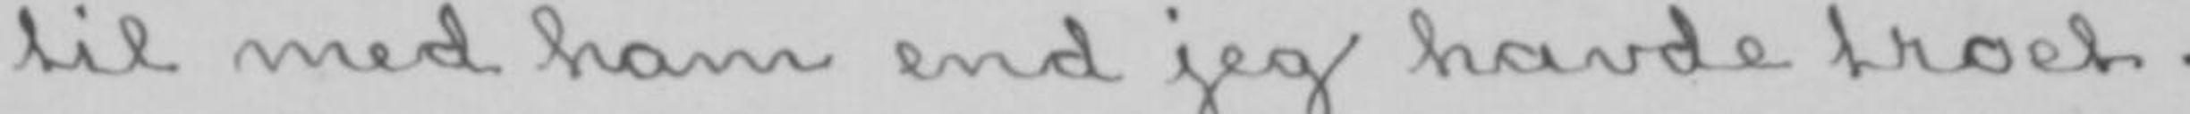til med ham end jeg havde troet.
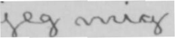jeg mig
